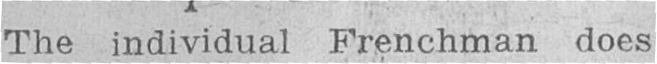The individual Frenchman does
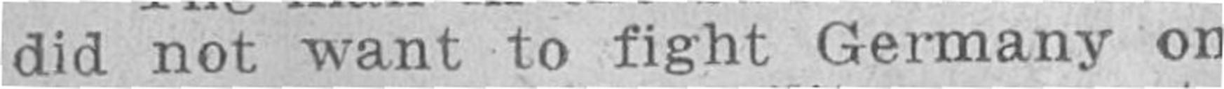did not want to fight Germany on
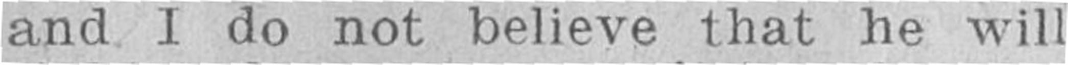and I do not believe that he will
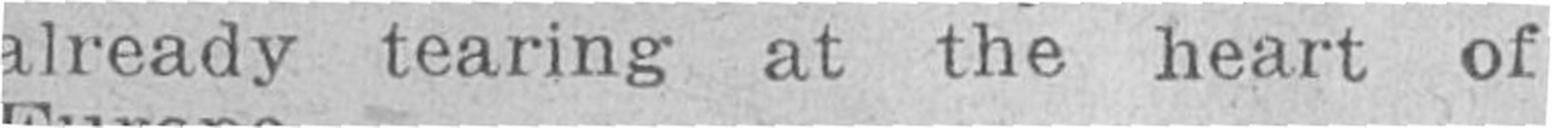already tearing at the heart of
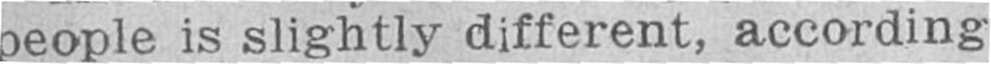people is slightly different, according
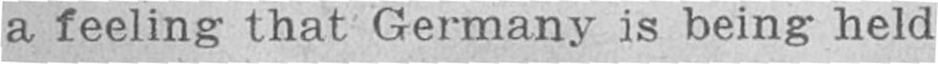a feeling that Germany is being held
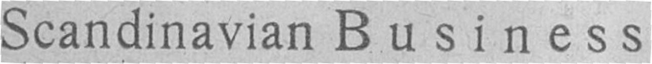Scandinavian Business
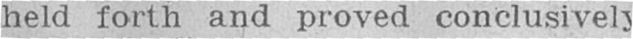held forth and proved conclusively
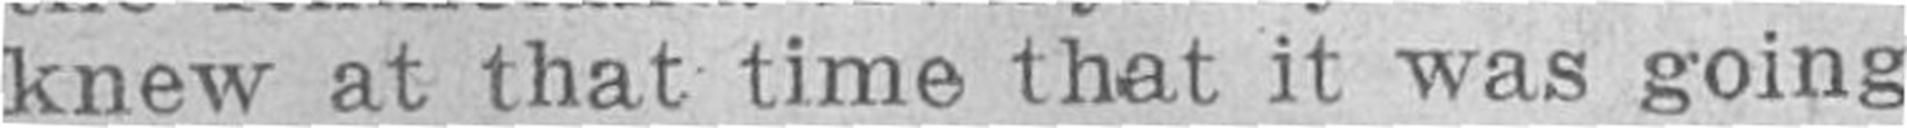knew at that time that it was going
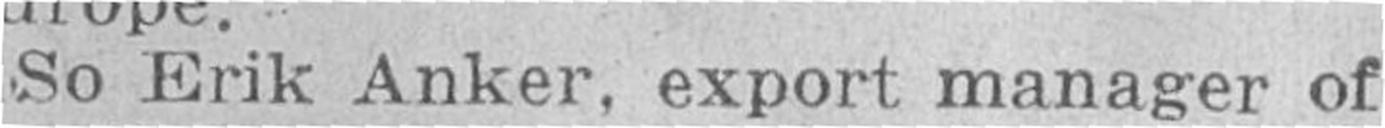So Erik Anker, export manager of
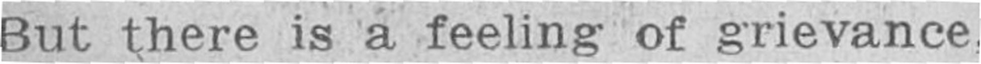But there is a feeling of grievance
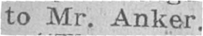to Mr. Anker.
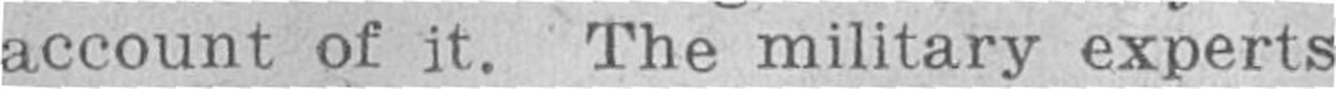account of it. The military experts
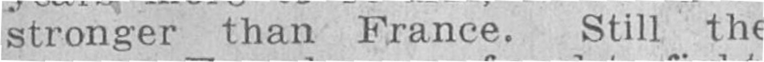stronger than France. Still the
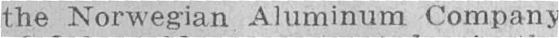the Norwegian Aluminum Company
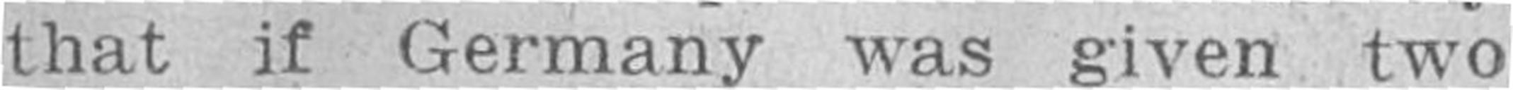that if Germany was given two
